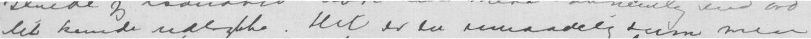let kunde udtrykke. Det er en umaadelig sum men
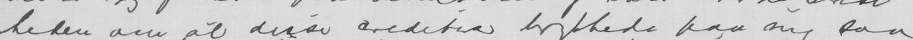heden om at disse creditia trykkede paa mig saa
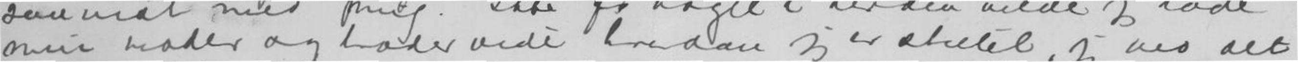min moder og broder vide hvordan jeg er stillet, jeg ved det
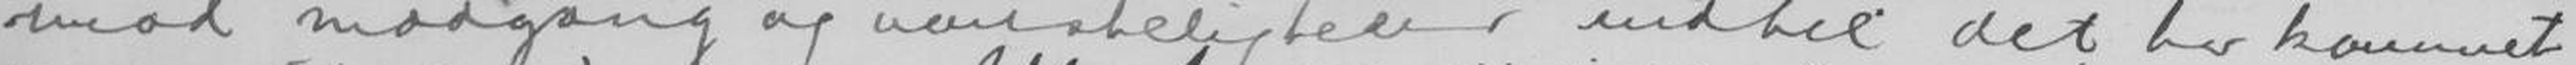mod modgang og vanskeligheder, indtil det har kommet
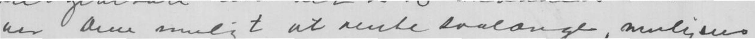var Dem muligt at vente saalænge, muligens
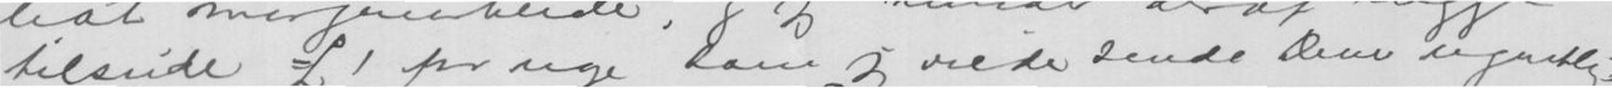tilside £1 pr uge som jeg vilde sende Dem ugentlig.
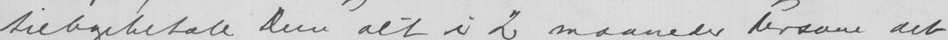tilbagebetale Dem alt i 2 maaneder dersom det
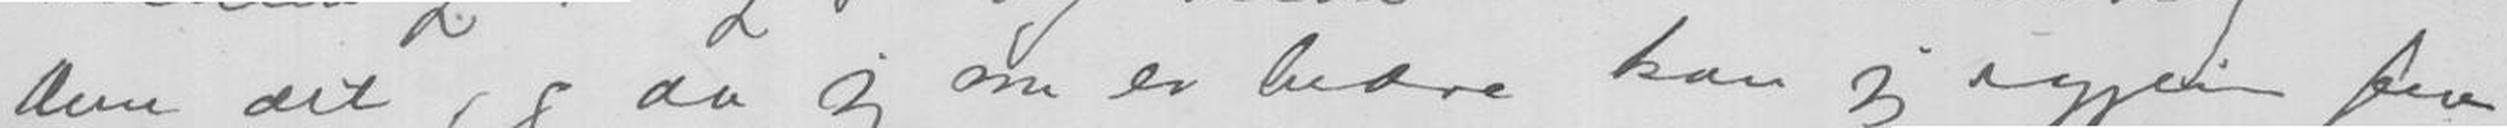Dem de, og da jeg nu er bedre kan jeg igjen faa
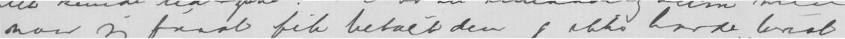naar jeg først fik betale den og ikke havde bevist-
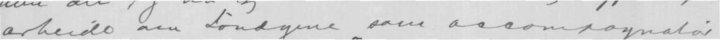arbeide om Lørdagene som accompagnatør
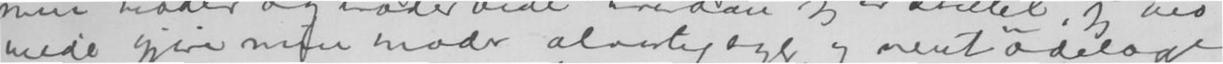vilde gjøre min moder alvorlig syg og rent ødelagt
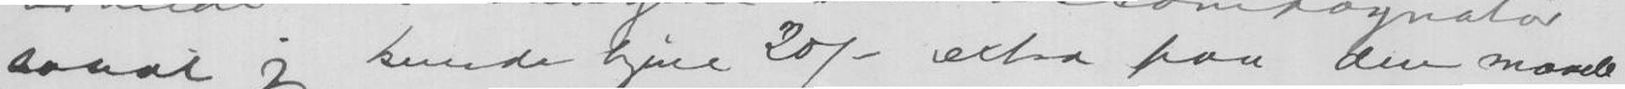saaat jeg kunde give 20/- extra paa den maade.
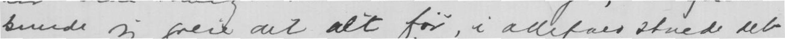kunde jeg greie det alt før, i allefald skulde det
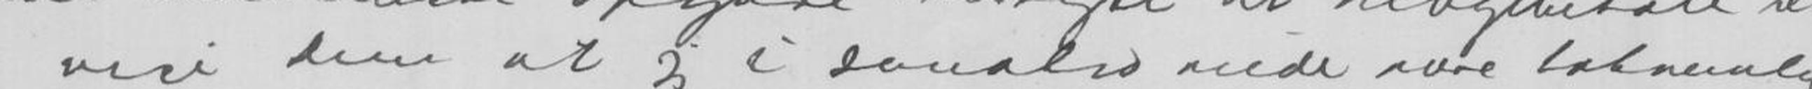og vise dem at jeg i sandhed vilde være taknemlig.
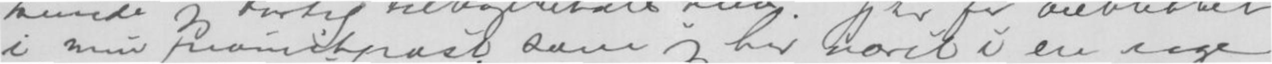i min Pianistpost som jeg har været i en uge
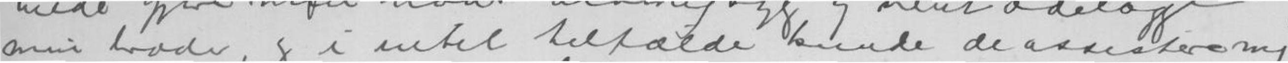min broder, og i enkel tilfælde kunde de assestere mig
